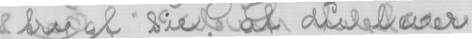trygt sie, at du lever
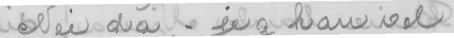Nei da, - jeg kan vel
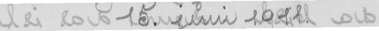15. juni 1911.
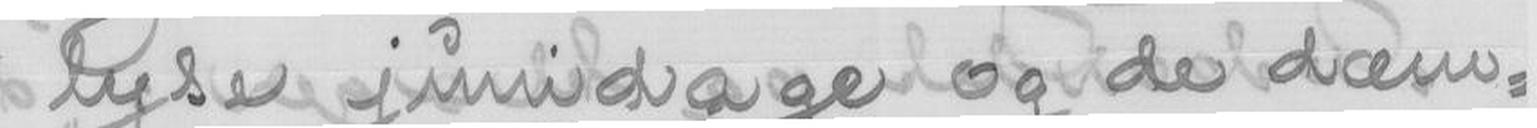lyse junidage og de dæm-
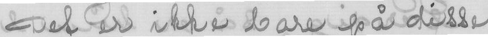Det er ikke bare på disse
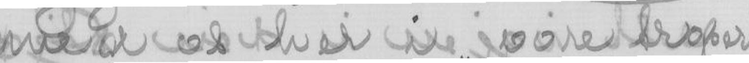med os her i "vore troper".
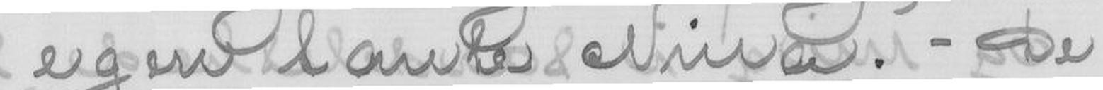egen tante Nina. - De
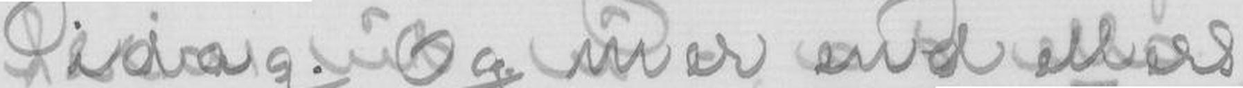idag. Og mer end ellers
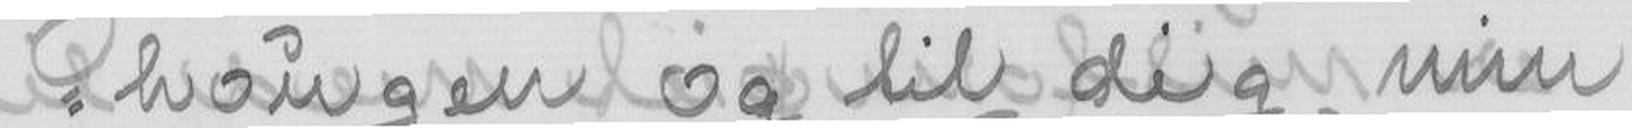hougen og til dig, min
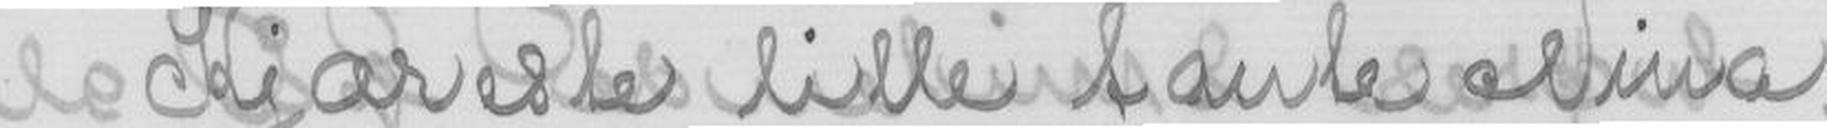Kjæreste lille tante Nina,
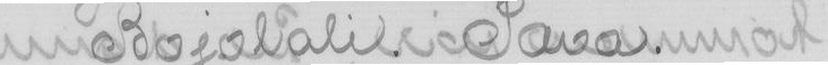Bojolali. Java.
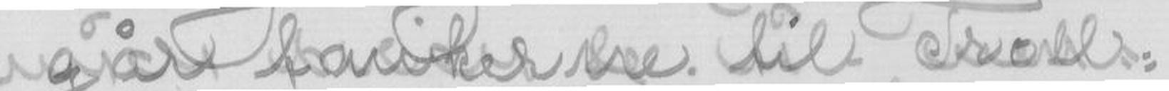går tankerne til Troll-
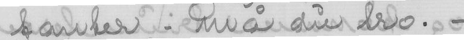tanker, - må du tro. -
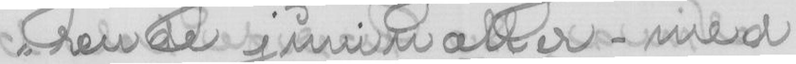rende juninætter - med
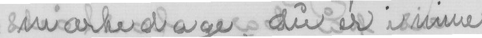mærkedage, du er i mine
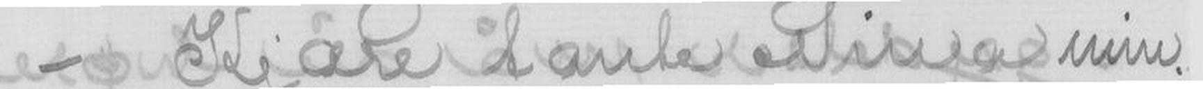Kjære tante Nina min.
